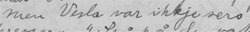Men Vesla var ikkje sers
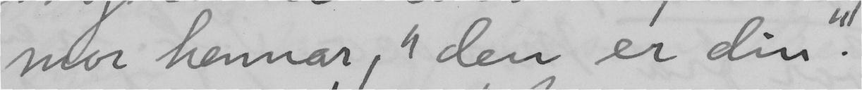mor hennar, "den er din".
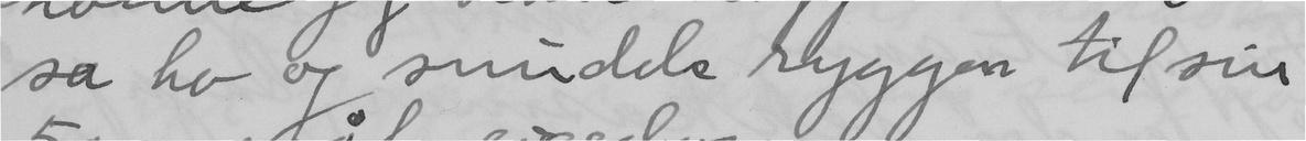sa ho og snudde ryggen til sin
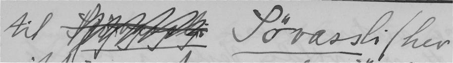til  Sövassli/hev
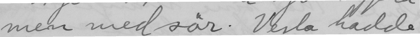men med sör. Vesla hadde
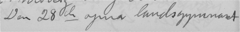Den 28de opna landsgymnaset
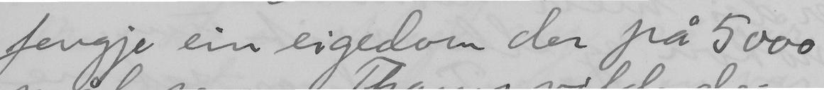fengje ein eigedom der på 5000
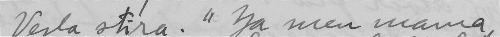Vesla stira. "Ja men mama,
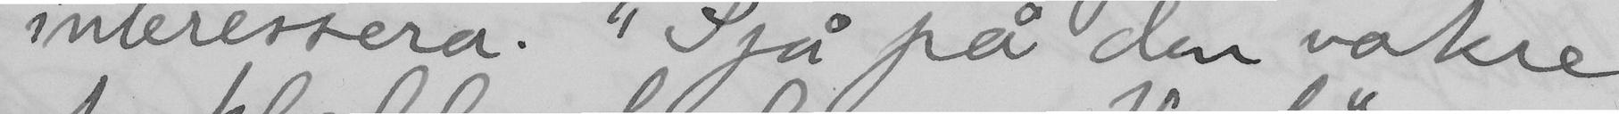interessera. "Sjå på den vakre
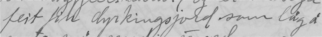feit fin dyrkingsjord som låg å
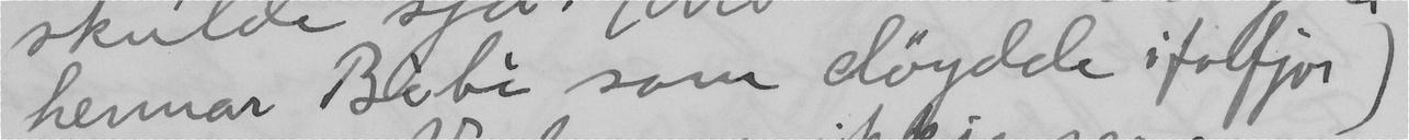hennar Bibi som döydde iforfjor)
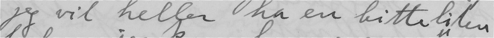jeg vil heller ha en bitteliten
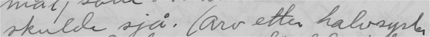skulde sjå. (Arv etter halvsyster
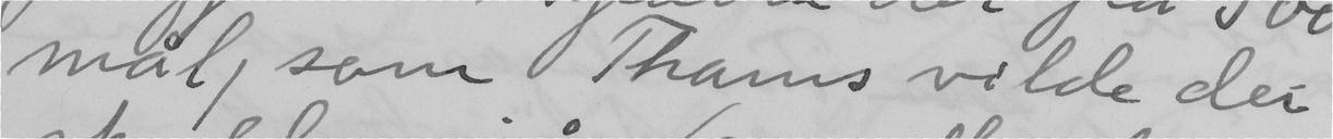mål, som Thams vilde dei
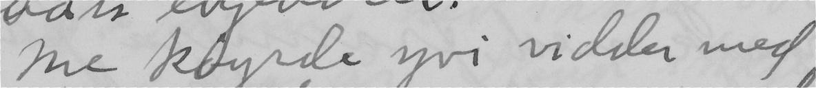Me köyrde yvi vidder med
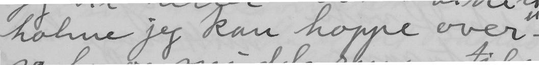holme jeg kan hoppe over" –
